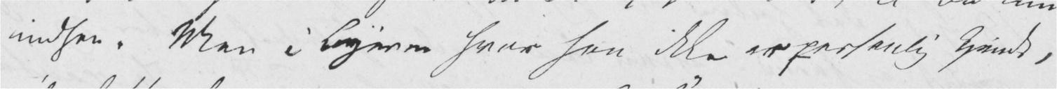indsee. Men i Byerne hvor han ikke er personlig kjendt,
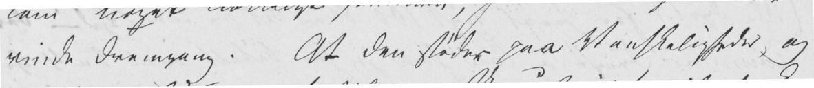vinde Fremgang. At den støder paa Vanskeligheder, og
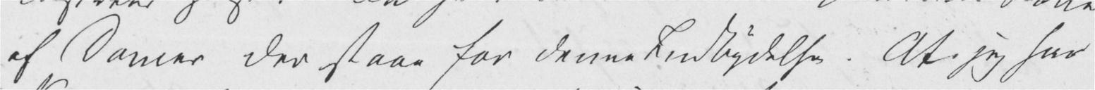af Damer der staae for denne Indbydelse. At jeg har
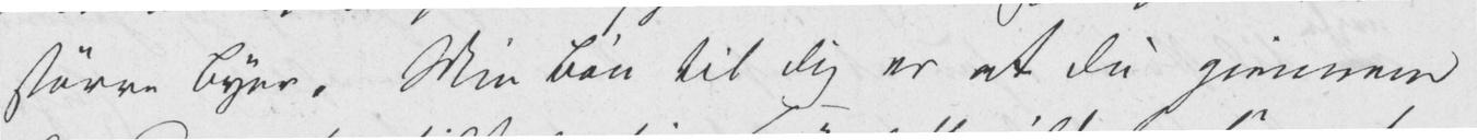større Byer. Min bøn til Dig er at Du gjennem
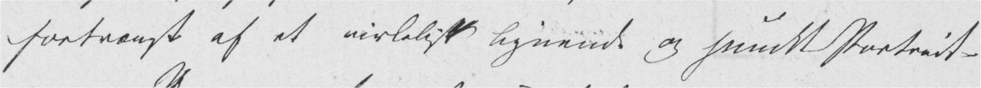fortrængt af et virkeligt lignende og smukt Portrait.
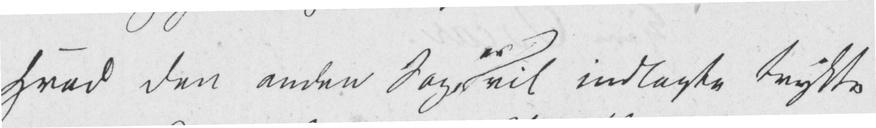Hvad den anden Sag er, vil indlagte trykte
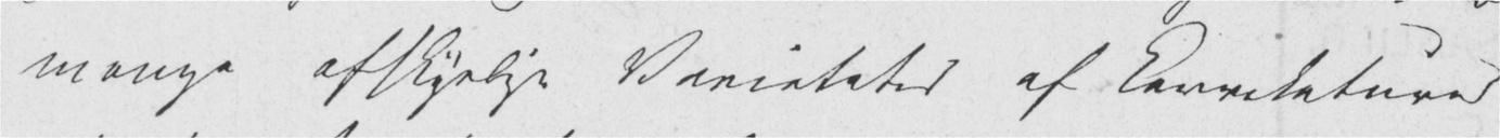mange afskyelige Varieteter af Karrikaturer
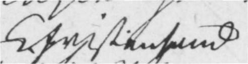Christiansand
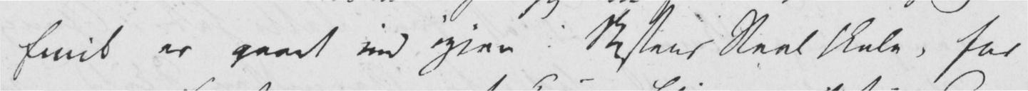Emil er gaaet ind igjen i Nissens Realskole, for
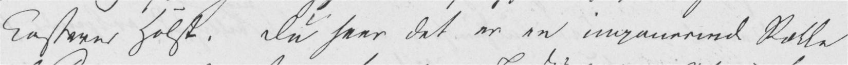Kasserer Holst. Du seer det er en imponerende Række
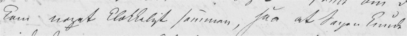kom noget klækkeligt sammen, saa at Sagen kunde
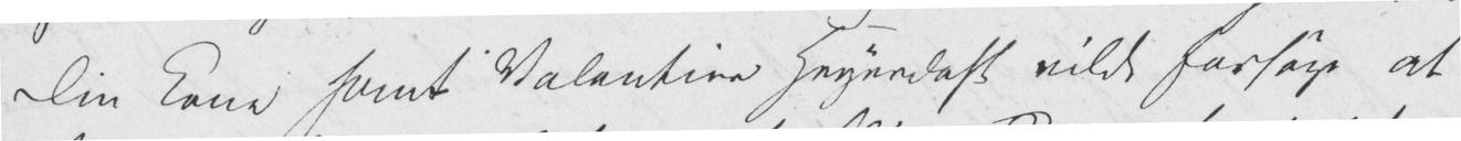Din Kone samt Valentine Heyerdahl vilde forsøge at
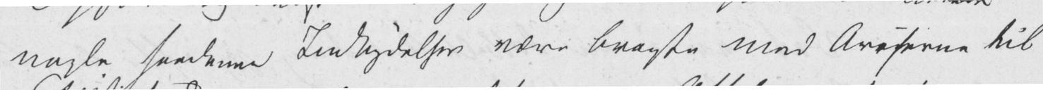nogle saadanne Indbydelser være bragte med Aviserne til
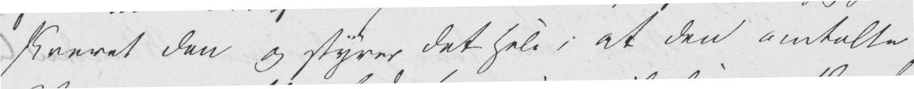skrevet den og styrer det Hele; at den omtalte
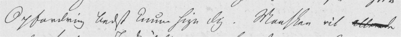Opfordring bedst kunne sige Dig. Maaske vil allerede
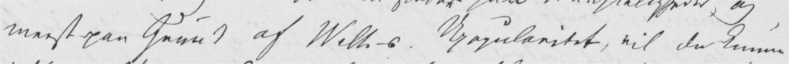meest paa Grund af Welh-s. Upopularitet, vil Du kunne
